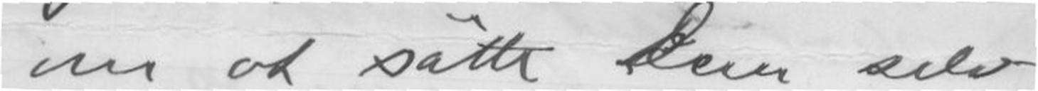om at sätte Dem selv
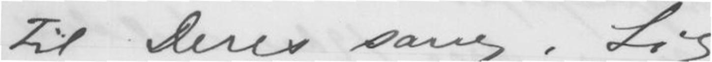til Deres sang. Sig
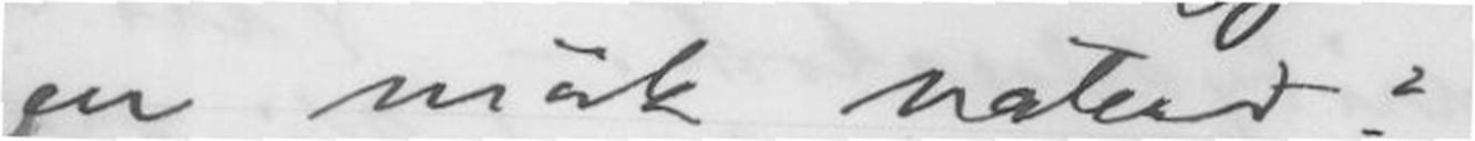en mörk natur?
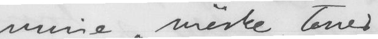mine mörke Toner
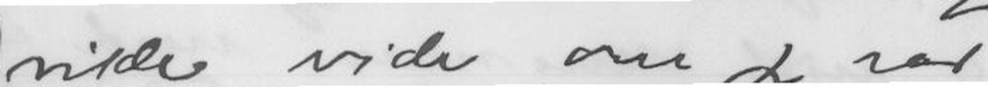vilde vide om jeg var
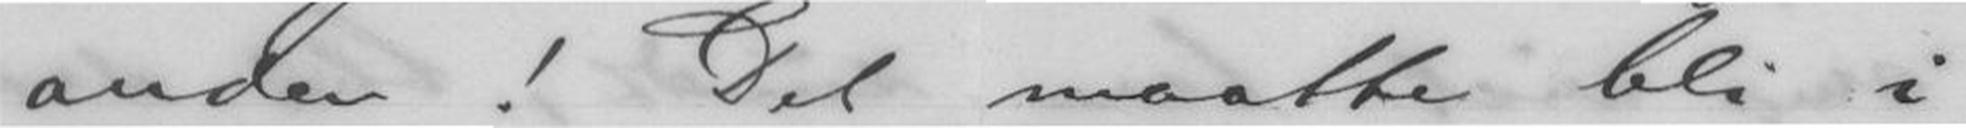anden! Det maatte bli i
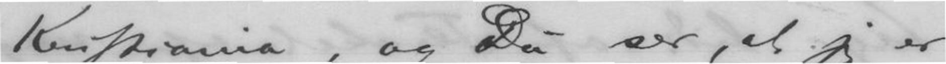Kristiania, og Du ser, at jeg er
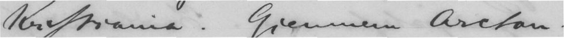Kristiania. Gjennem Arctan-
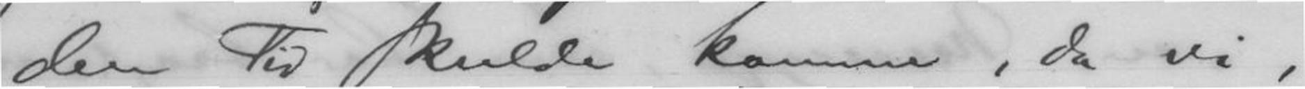den Tid skulde komme, da vi,
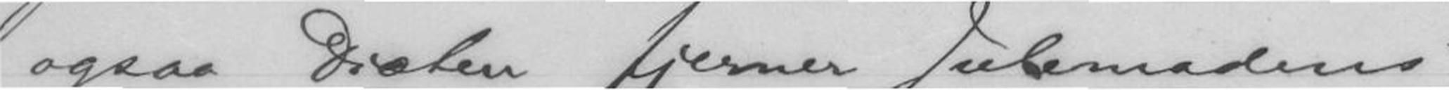ogsaa Diæten fjerner Julemadens
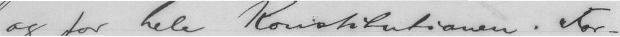og for hele Konstitutionen. For-
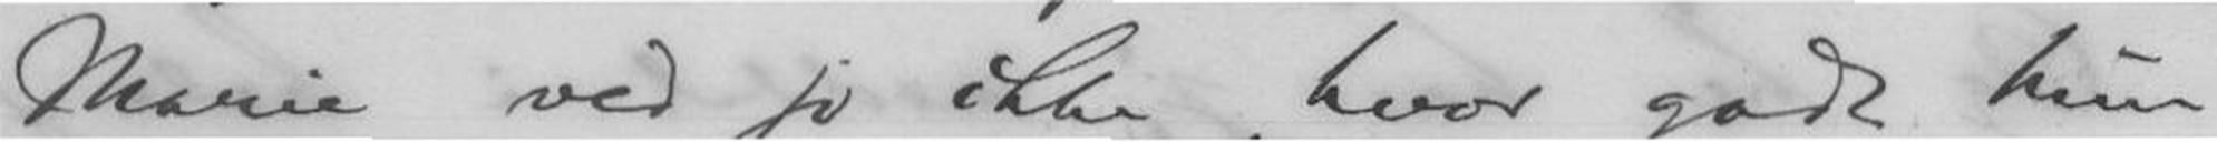Marie ved jo ikke, hvor godt hun
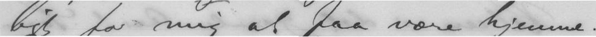ligt for mig at faa være hjemme.
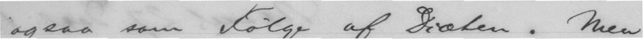ogsaa som Følge af Diæten. Men
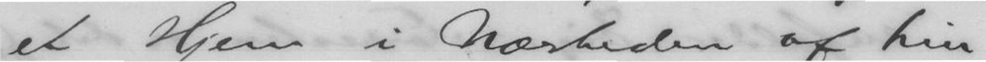et Hjem i Nærheden af hin-
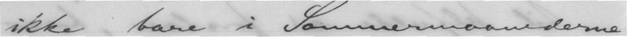ikke bare i Sommermaanederne,
